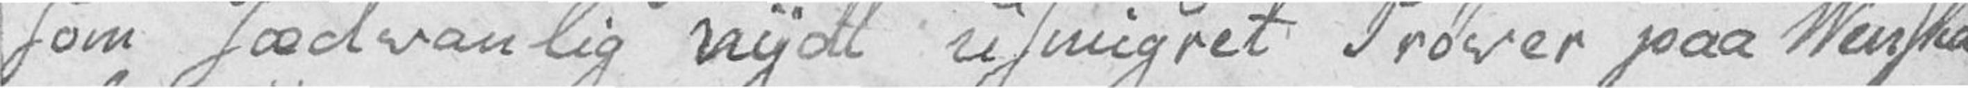som sædvanlig nydt usmigret Prøver paa Venska
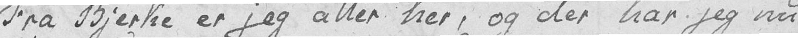Fra Bjerke er jeg atter her, og der har jeg nu
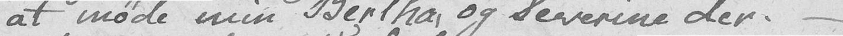at møde min Bertha og Severine der. –
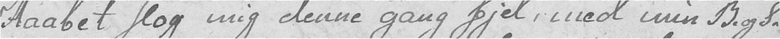Haabet slog mig denne gang fjel, med min B. og S.
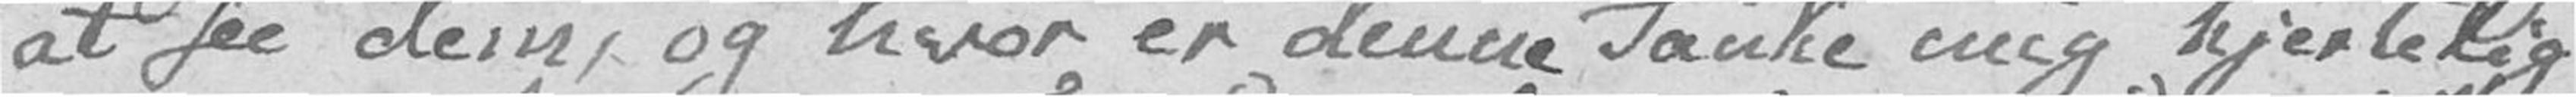at see dem, og hvor er denne Tanke mig hjertelig
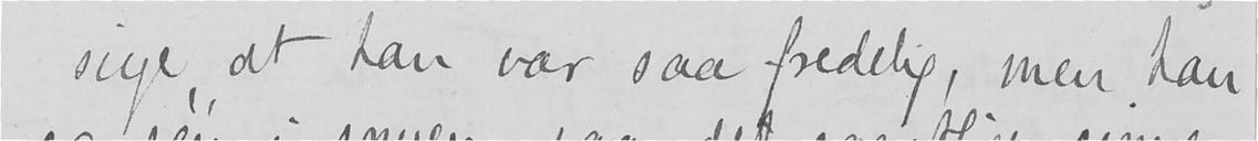sige, at han var saa fredelig, men han
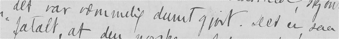det var væmmelig dumt gjort. Det er saa
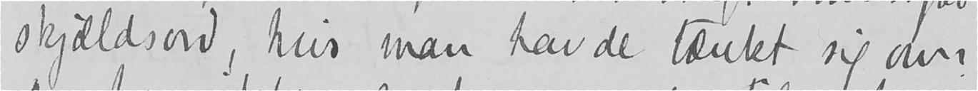skjældsord, hvis man havde tænkt sig om?
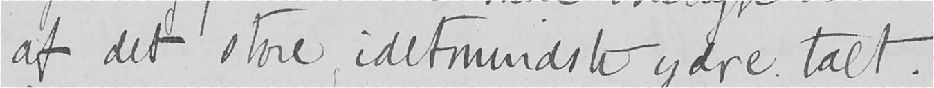af det store, idetmindste ydre talt.
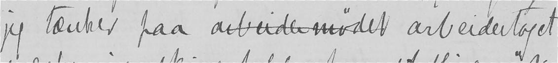jeg tænker paa arbeidermødet arbeidertoget
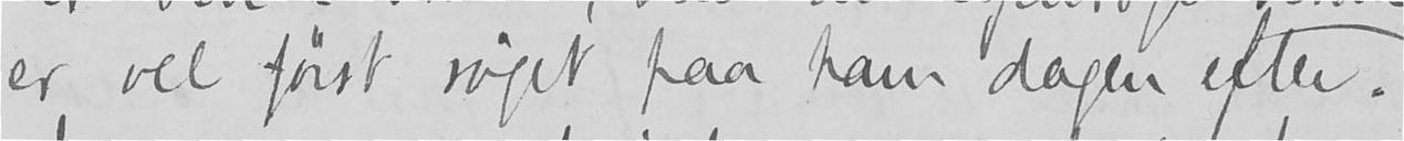er vel først røget paa ham dagen efter.
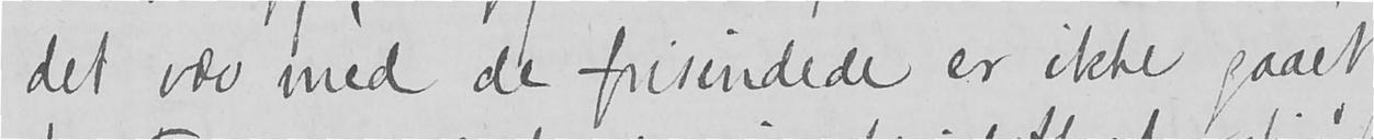det væv med de frisindede er ikke gaaet
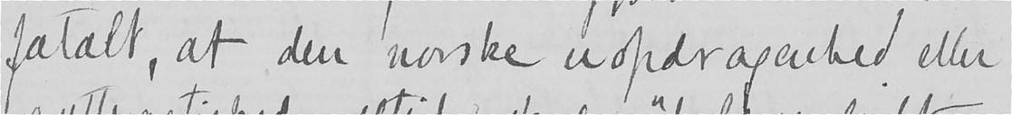fatalt, at den norske uopdragenhed eller
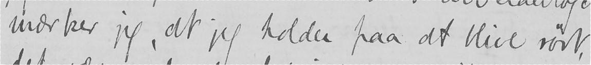mærker jeg, at jeg holder paa at blive rørt,
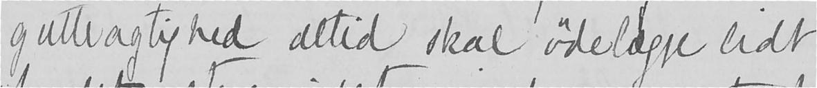gutteagtighed altid skal ødelægge lidt
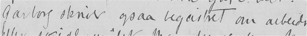Garborg skriver ogsaa begeistret om arbeids
